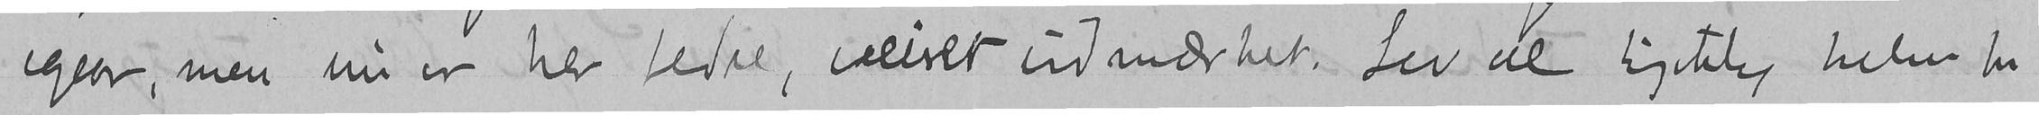igaar, men nu er her bedre, veiret udmærket. Lev vel hjertelig hilsen din
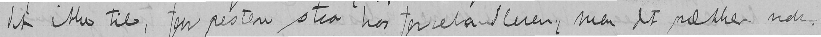det ikke til, faar resten staa hos farvehandleren, men det rækker nok.
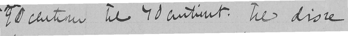90 centimer til 70 centimet. Til disse
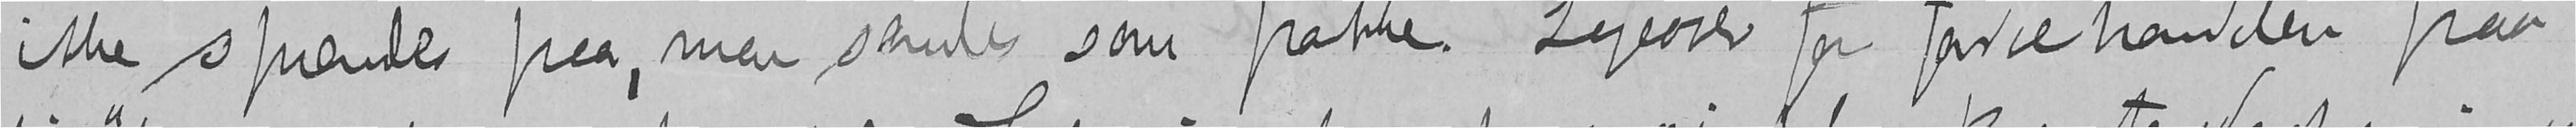ikke spændes paa, men sendes som pakke. Ligeover for farvehandelen paa
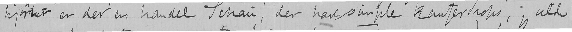hjørnet er der en handel Schau, der have simple kamferdrops, jeg vilde
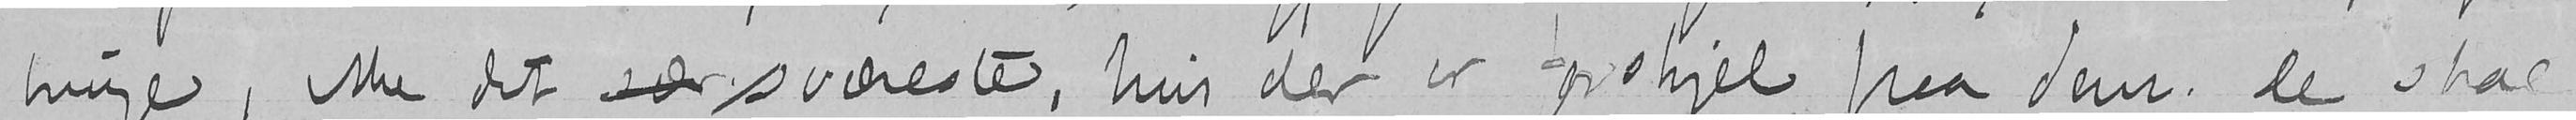bringe, ikke det særsværeste, hvis der er forskjel paa dem. De skal
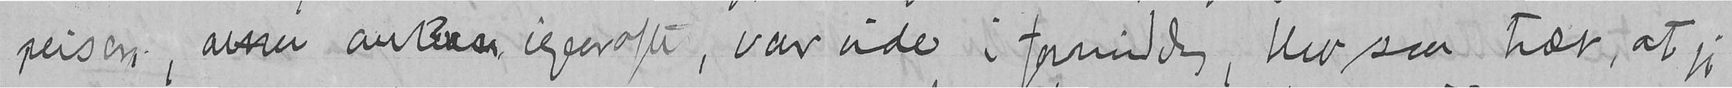reisen, anse anternn igaaraften, var ude i fomiddag, blev saa træt, at jeg
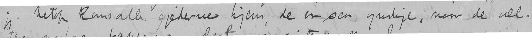jeg. Netop kom alle gjeterne hjem, de er saa grundige, naar de vel-
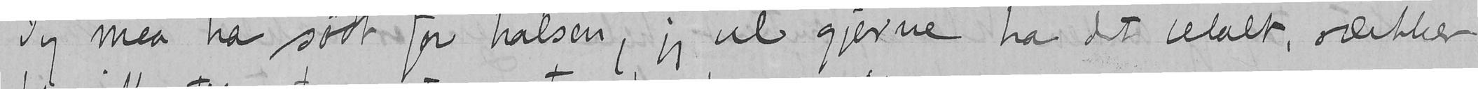Jeg maa ha sødt for halsen, jeg vil gjerne ha det betalt, rækker
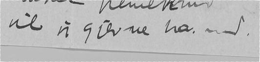vil jeg gjerne ha med.
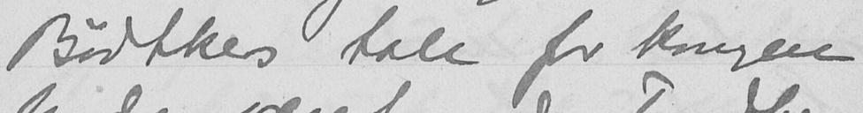Bødtkers tale for kongen
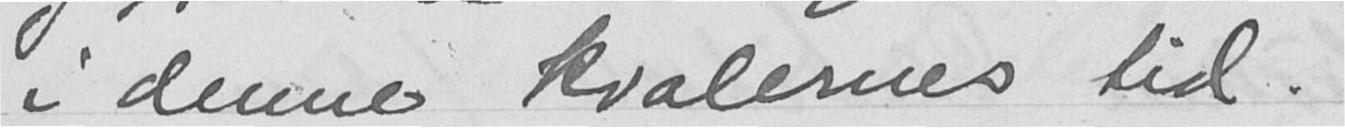i denne kvalernes tid.
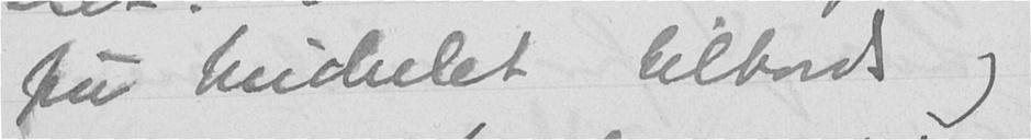fru Michelet tilbords og
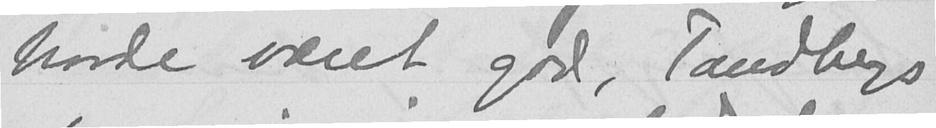havde været god, Tandbergs
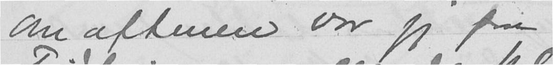Om aftenen var jeg paa
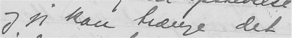og jeg kan trænge det
